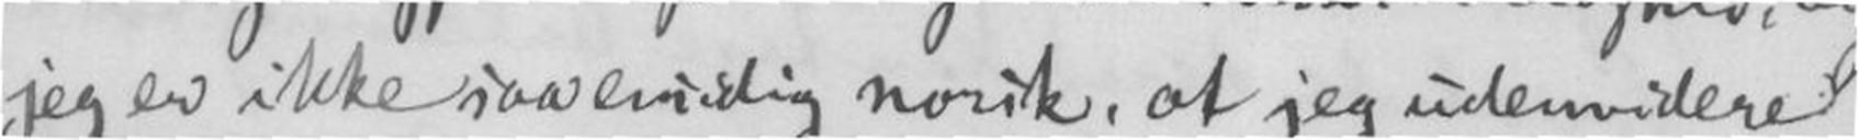jeg er ikke saa ensidig norsk, at jeg udenvidere
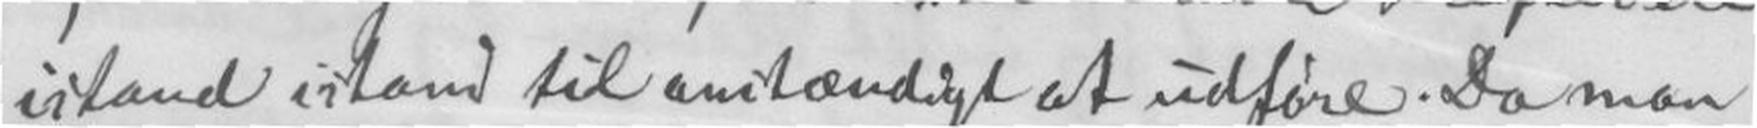istand istand til anstændigt at udføre. Da man
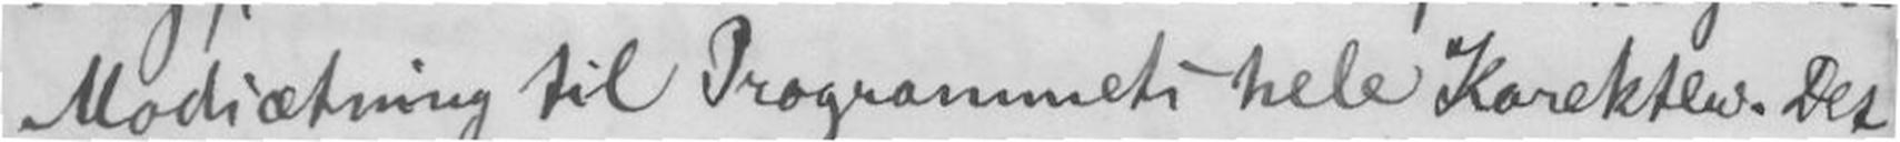Modsætning til Programmets hele Korekter. Det
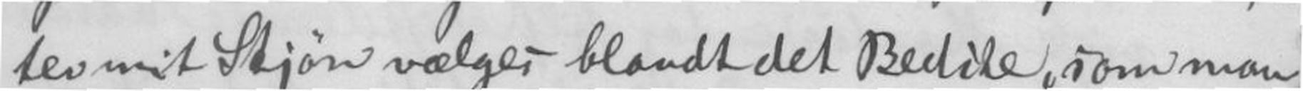ter mit Skjøn vælges blandt det Bedste, som man
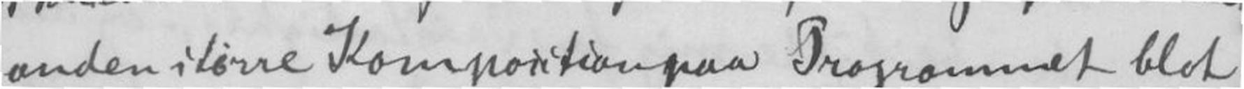anden større Komposition paa Programmet blot
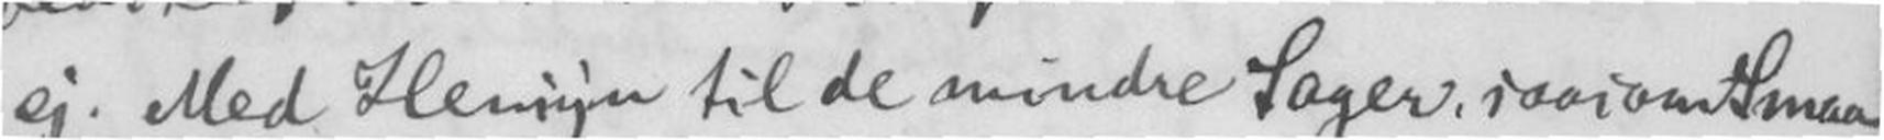ej. Med Hensyn til De mindre Sager saa som Smaa-
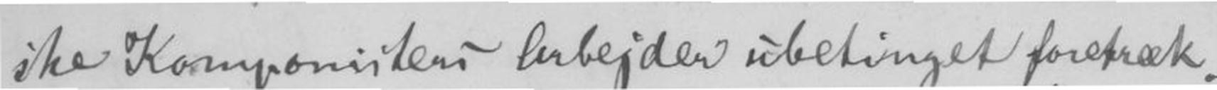ske Komponisters Arbejder ubetinget foretræk.
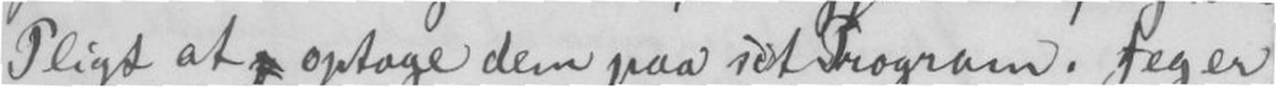Pligt at optage dem paa sit Program. Jeg er
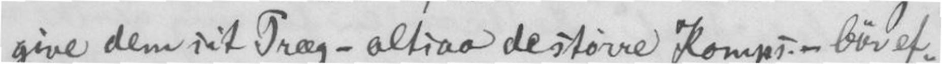give dem sit Præg - altsaa destørre Komps. - bør ef-
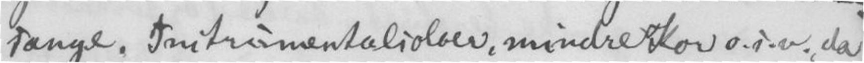sange, Initrumentalsolver, mindre Kor o.s.v., da
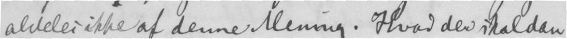aldeles ikke af denne Mening. Hvad der skal dan-
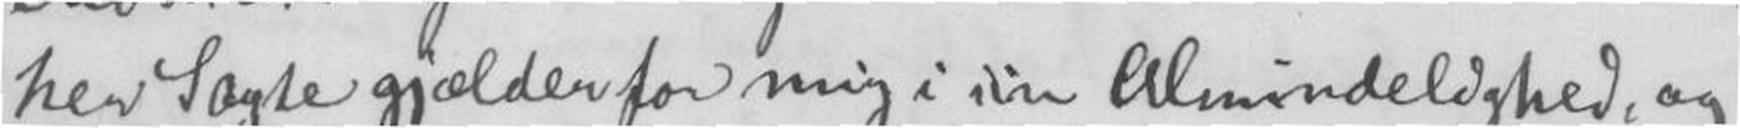her Sagte gjældert for mig i sin Almindelighed, og
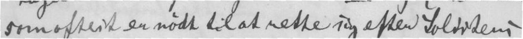som oftes er nødt til at rette sig efter Solistens
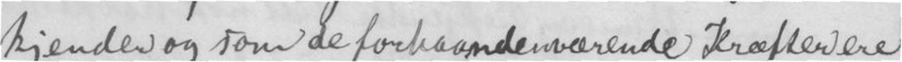kjender og som de forhaanden værende Kræfter ere
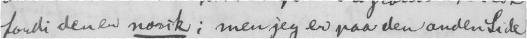fordi den er norsk; men jeg er paa den anden Side
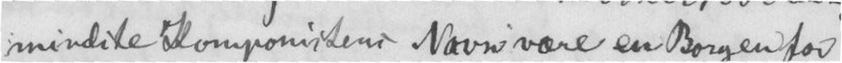mindste Komponistens Navn være en Borgen for
